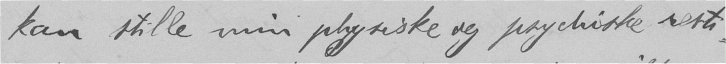kan stille min physiske og psychiske resti-
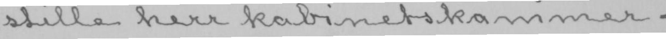stille herr kabinetskammer-
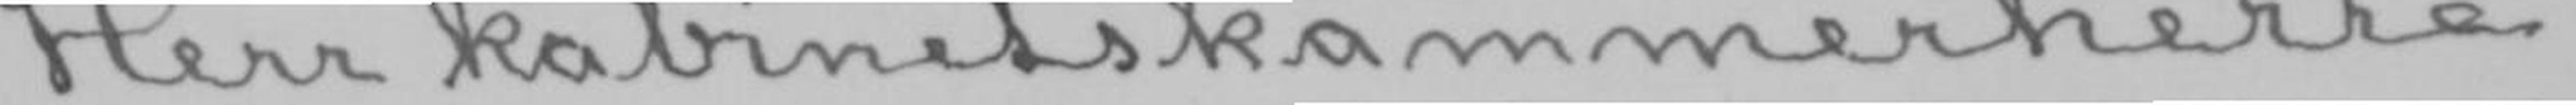Herr kabinetskammerherre
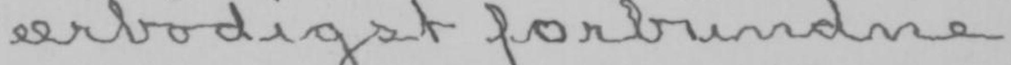ærbødigst forbundne
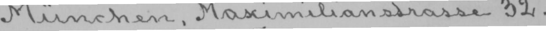München, Maximilianstrasse 32.
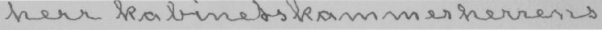herr kabinetskammerherrens
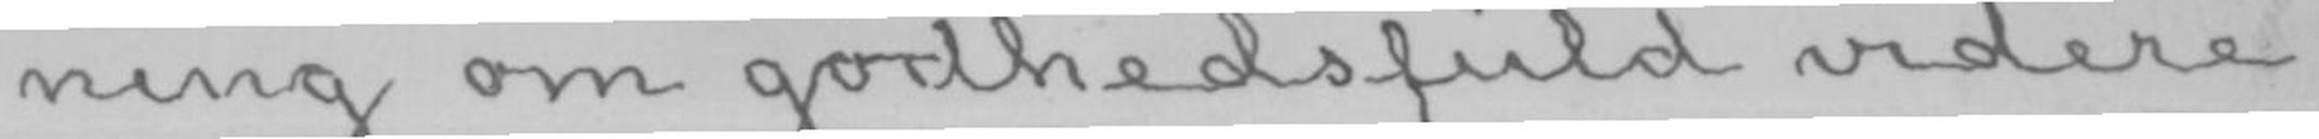ning om godhedsfuld videre
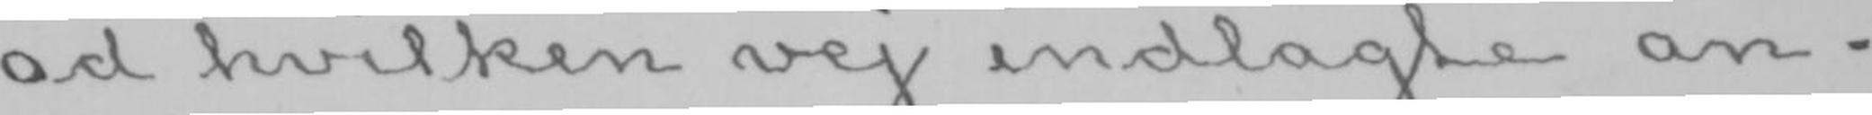ad hvilken vej indlagte an-
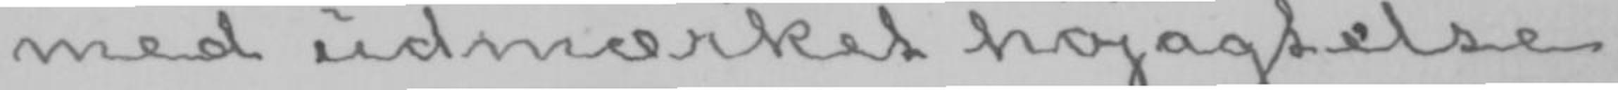med udmærket højagtelse
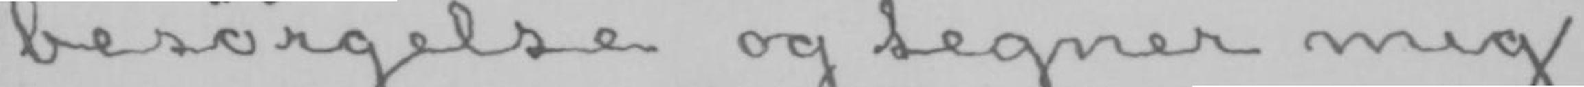besørgelse og tegner mig
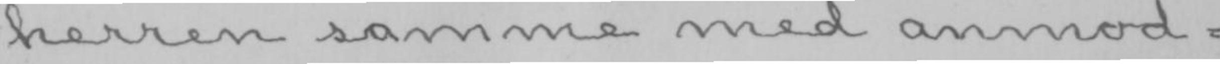herren samme med anmod-
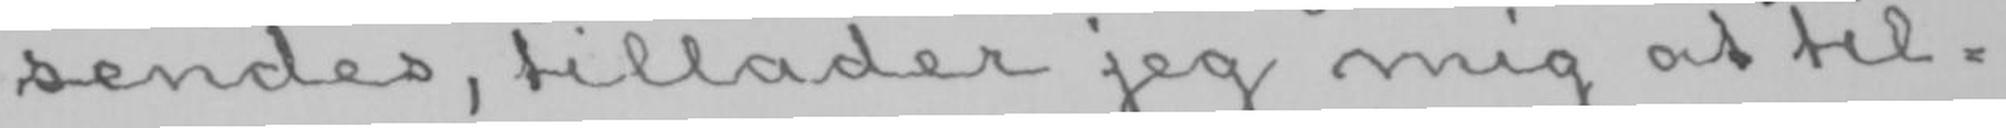sendes, tillader jeg mig at til-
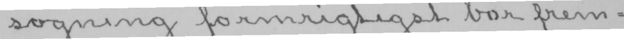søgning formrigtigst bør frem-
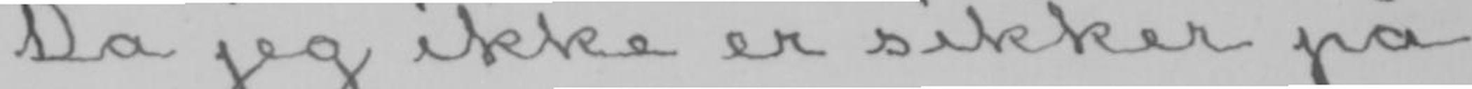Da jeg ikke er sikker på
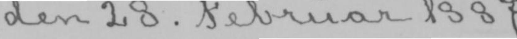den 28. Februar 1887.
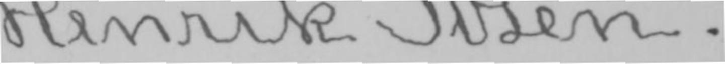Henrik Ibsen.
